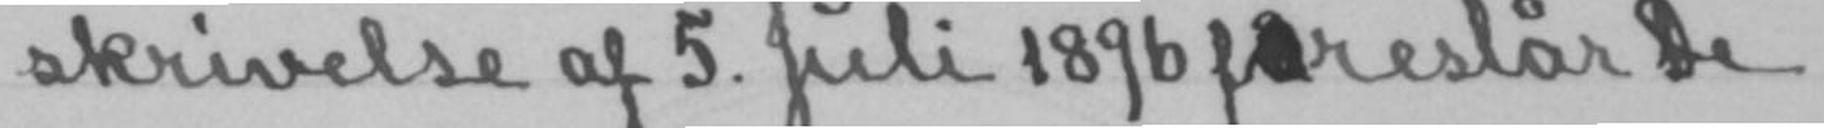skrivelse af 5. Juli 1896 foreslår De
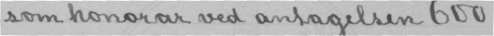som honorar ved antagelsen 600
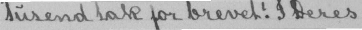Tusend tak for brevet! I Deres
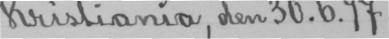Kristiania, den 30.6.97.
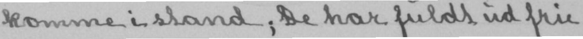komme i stand; De har fuldt ud frie
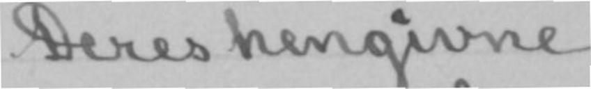Deres hengivne
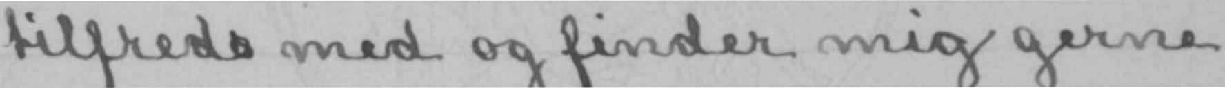tilfreds med og finder mig gerne
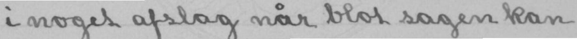i noget afslag når blot sagen kan
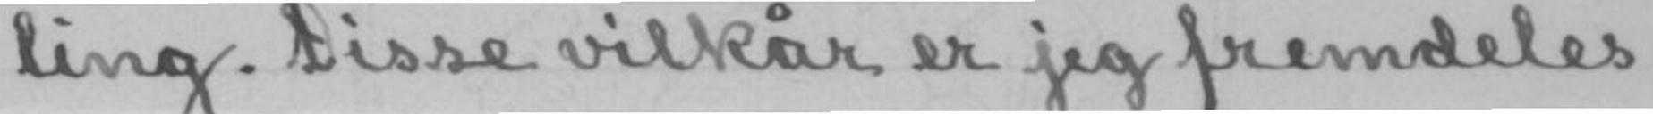ling. Disse vilkår er jeg fremdeles
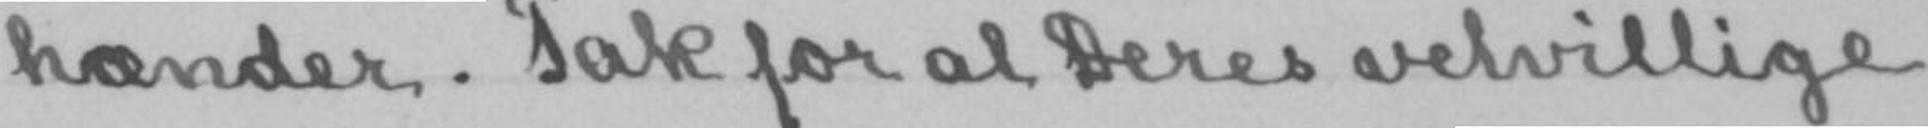hænder. Tak for al Deres velvillige
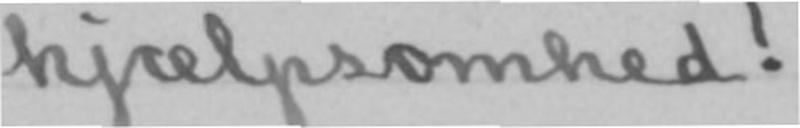hjælpsomhed!
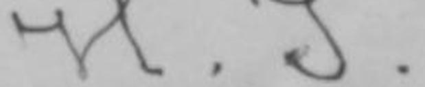H. I.
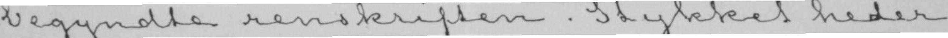begyndte renskriften. Stykket heder
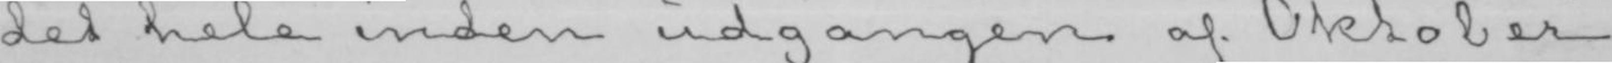det hele inden udgangen af Oktober;
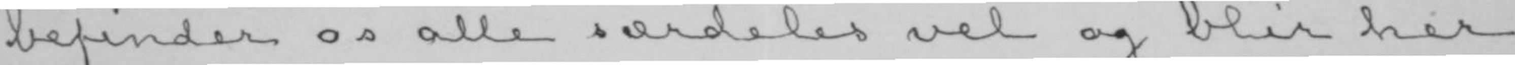befinder os alle særdeles vel og blir her
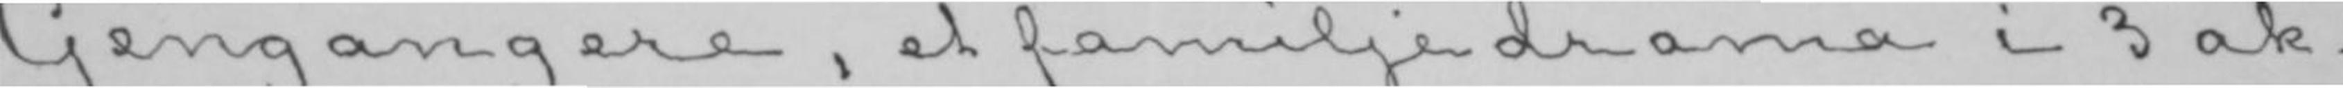Gengangere, et familjedrama i 3 ak-
